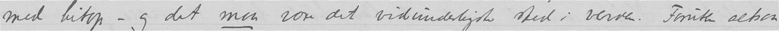med hitop - og det maa være det vidunderligste sted i verden. Foruten altsaa
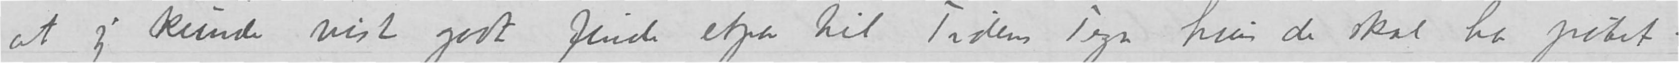at jeg kunde vist godt finde etpar til Tidens Tegn hvis de skal ha potet -
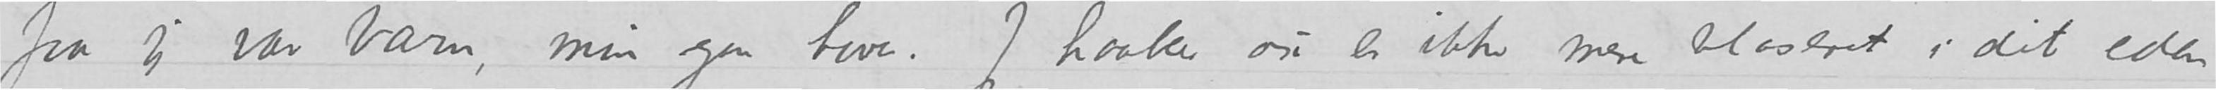fra jeg var barn, min egen have. Jeg haaber du er ikke mere blaseret i dit eden
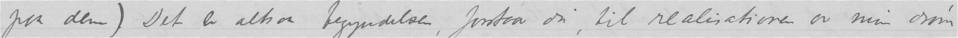paa dem) Det er altsaa begyndelsen, forstaar du, til realisationen av min drøm
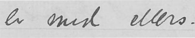er med ellers.
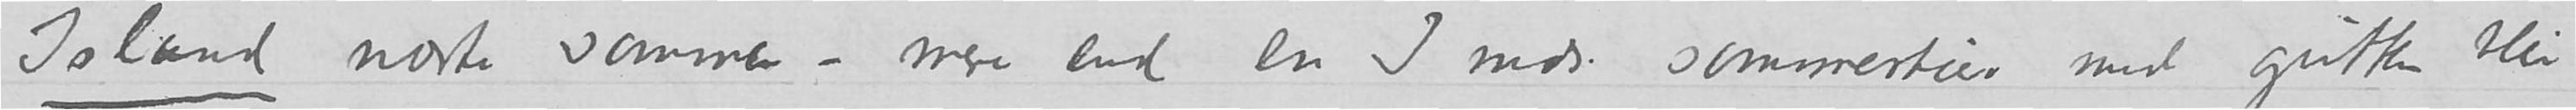Island næste sommer - mere end en 1 mds. sommerstur med guttene blir
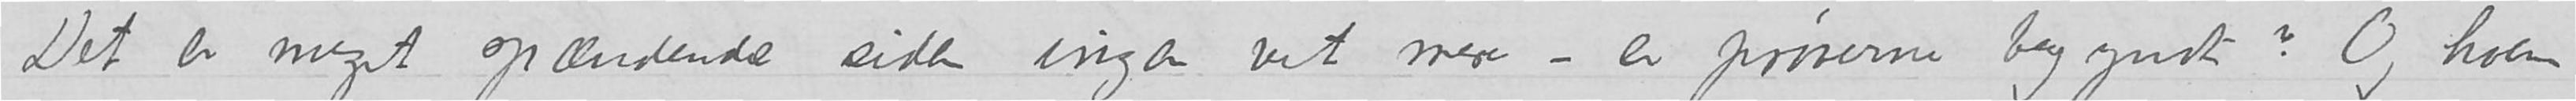Det er noget spændende siden ingen vet mere - er prøverne begyndt? Og hvem
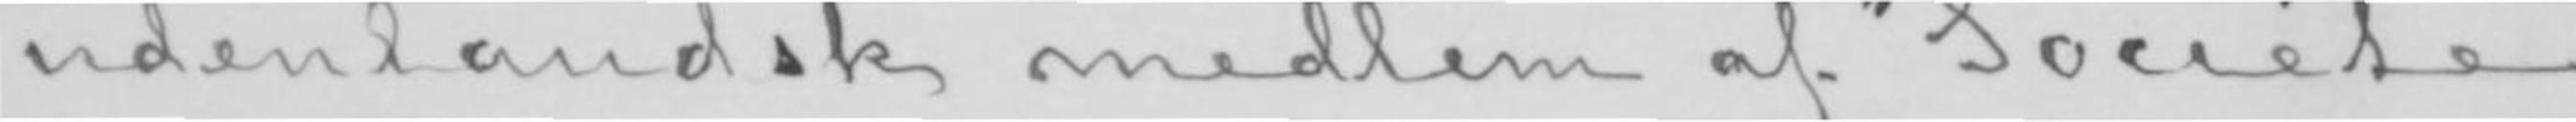udenlandsk medlem af «Société
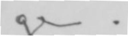ge.
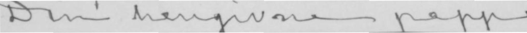Din hengivne pappa
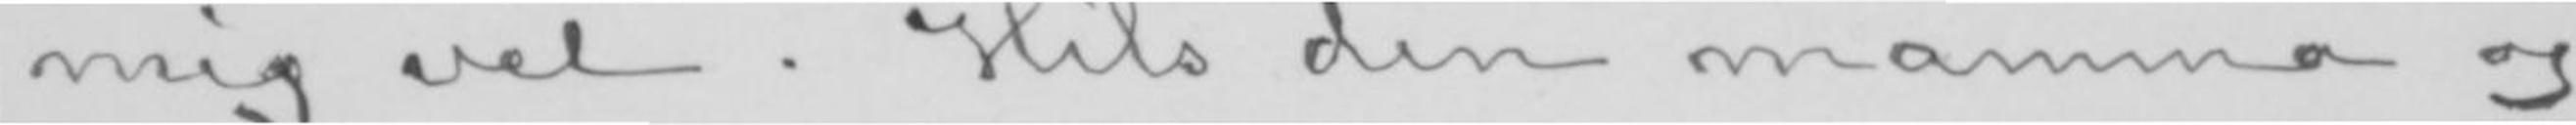mig vel. Hils din mamma og
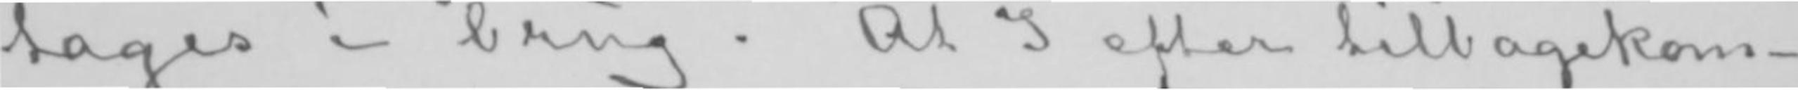tages i brug. At I efter tilbagekom-
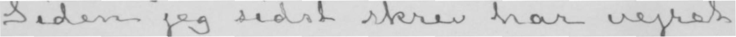Siden jeg sidst skrev har vejret
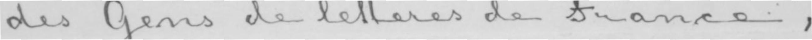des Gens de letteres de France»,
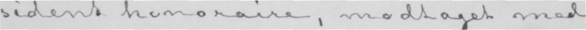sident honoraire, modtaget med-
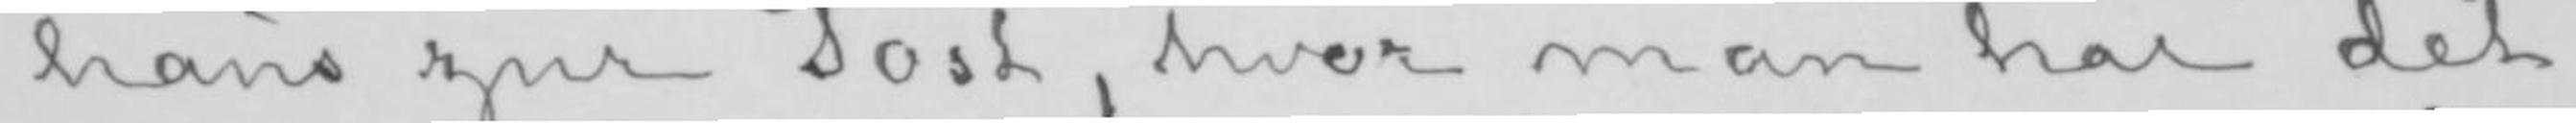haus zur Post, hvor man har det
